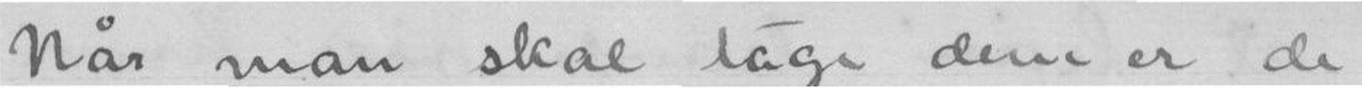Når man skal lage dem er de
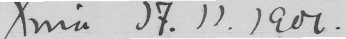Xania 17.11.1901
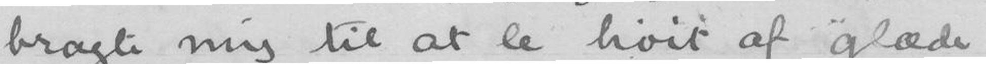bragte mig til at le høit af glæde
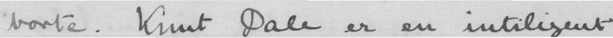borte. Knut Dale er en inteligent
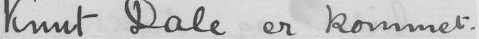Knut Dale er kommet.
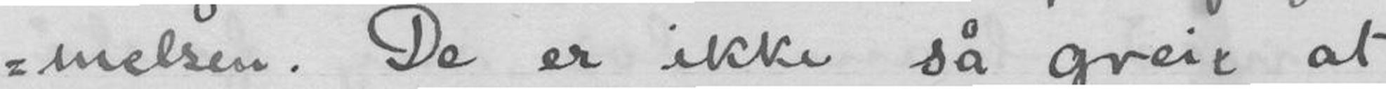melsen. De er ikke så greie at
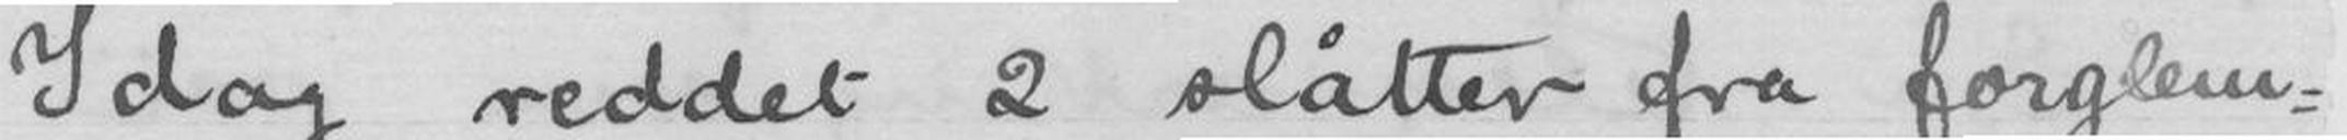Idag reddet 2 slåtter fra forglem-
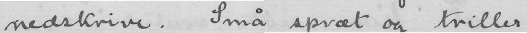nedskrive. Små spræt og triller
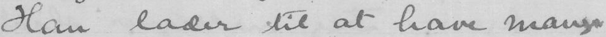Han lader til at have mange
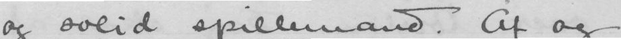og solid spillemand. Af og
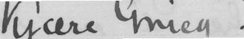Kjære Grieg!
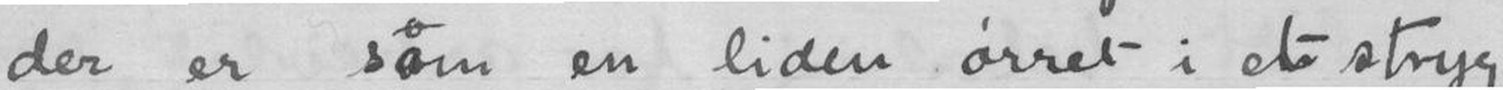der er som en liden ørret i et stryg.
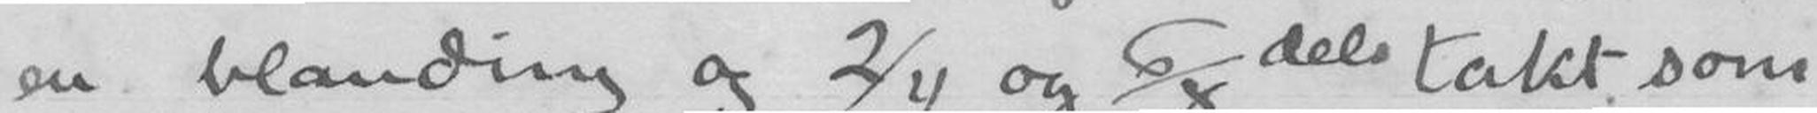en blanding af 2/4 og 6/8 dels takt som
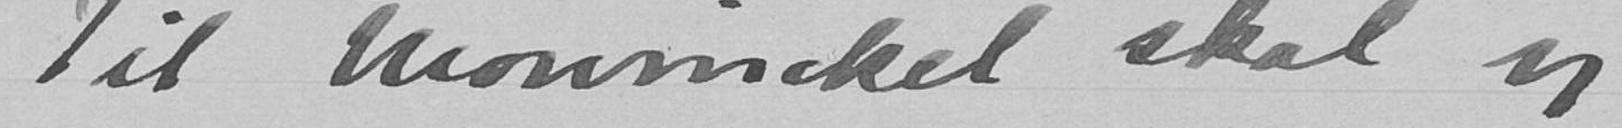Til Mowinkel skal jeg
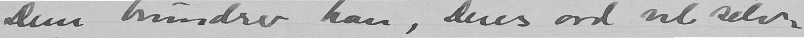Dem beundrer han, Deres ord vil selv-
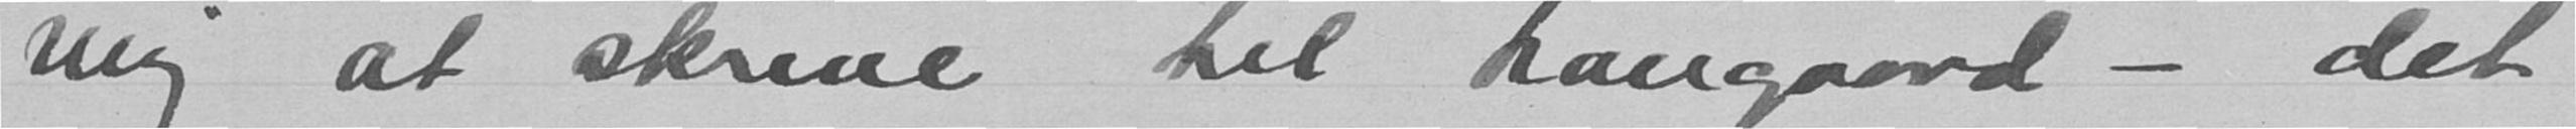mig at skrive til Langaard - det
